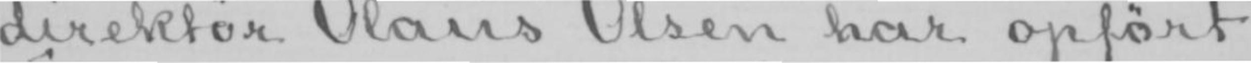direktør Olaus Olsen har opført
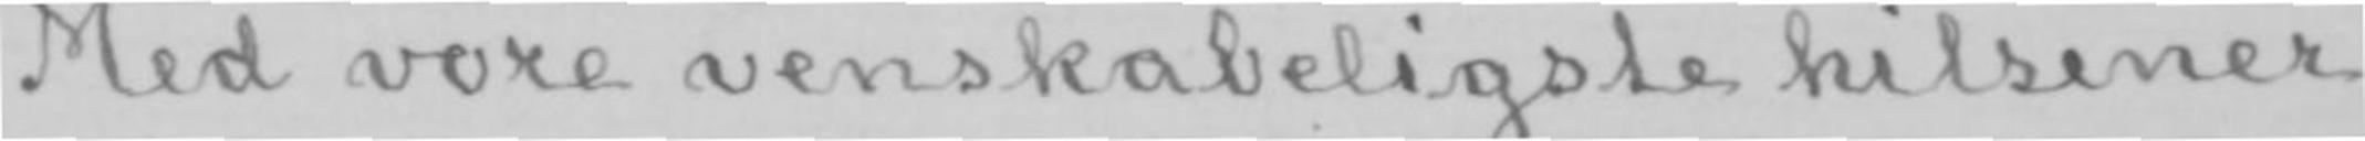Med vore venskabeligste hilsener
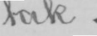tak.
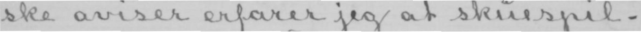ske aviser erfarer jeg at skuespil-
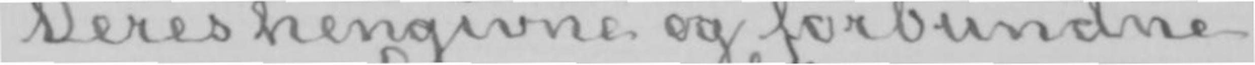Deres hengivne og forbundne
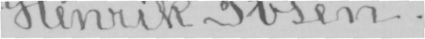Henrik Ibsen.
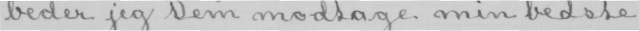beder jeg Dem modtage min bedste
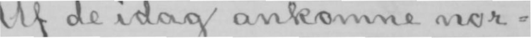Af de idag ankomne nor-
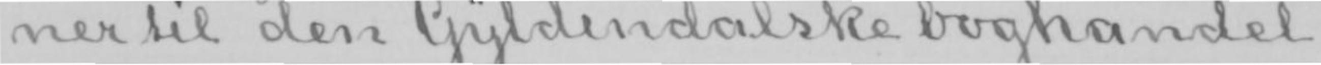ner til den Gyldendalske boghandel
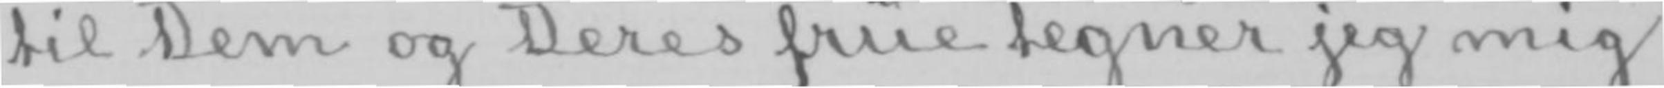til Dem og Deres frue tegner jeg mig
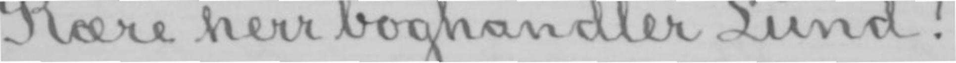Kære herr boghandler Lund!
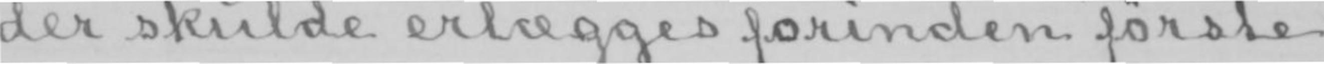der skulde erlægges forinden første
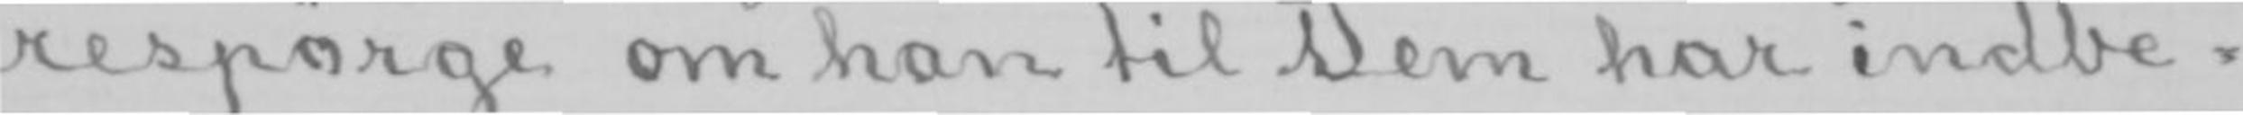respørge om han til Dem har indbe-
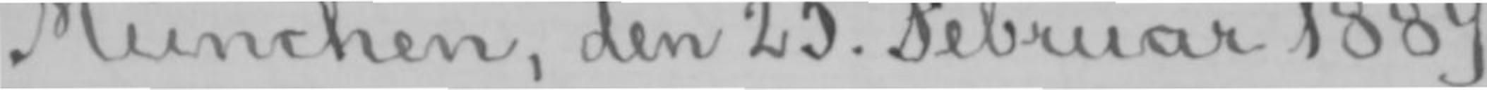München, den 25. Februar 1889.
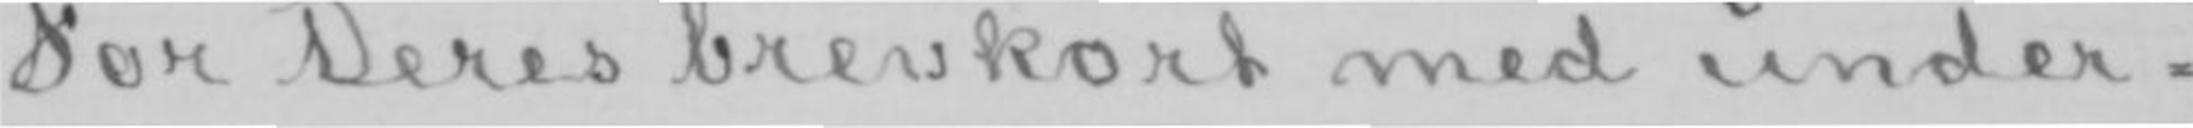For Deres brevkort med under-
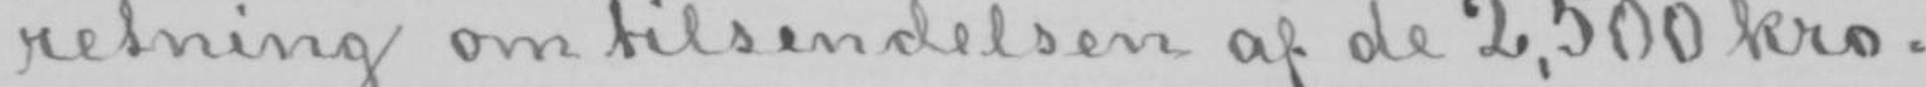retning om tilsendelsen af de 2,500 kro-
